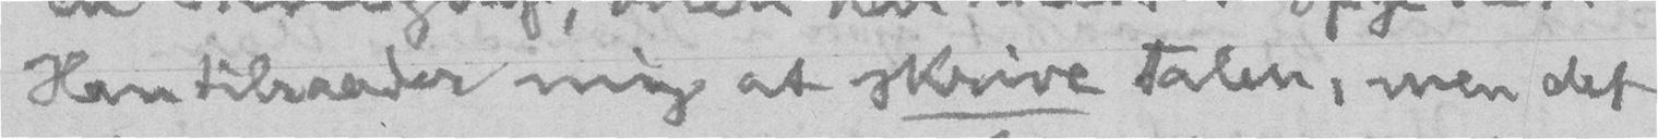Han tilraader mig at skrive Talen, men det
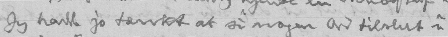Jeg hadde jo tænkt at si nogen Ord tilslut i
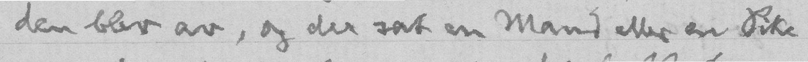den blev av, og der sat en Mand eller en Pike
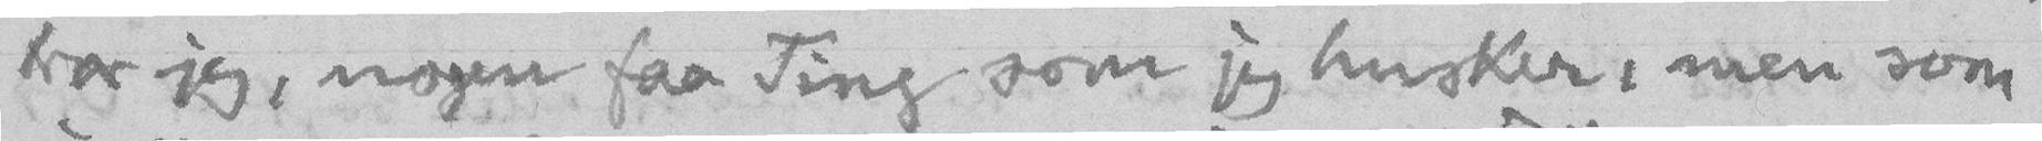tror jeg, nogen faa Ting som jeg husker, men som
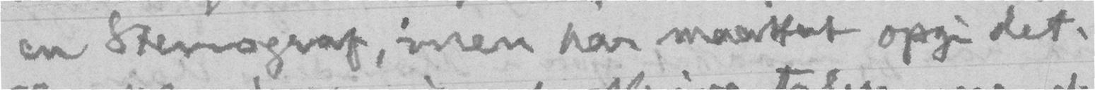en Stenograf, men har maattet opgi det.
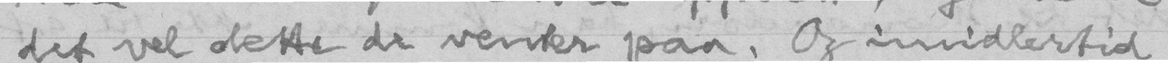det vel dette de venter paa. Og imidlertid
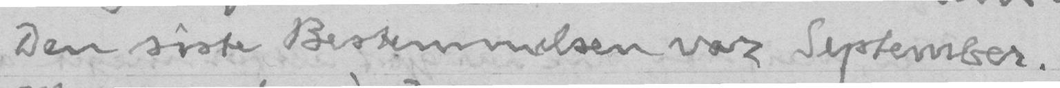Den siste Bestemmelsen var September.
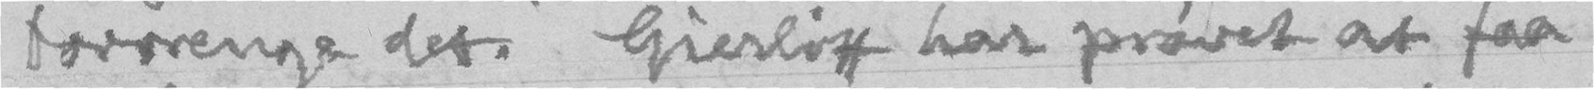forvrenge det. Gierløff har prøvet at faa
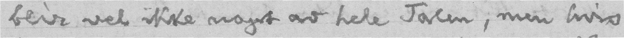blir vel ikke noget av hele Talen, men hvis
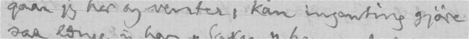gaar jeg her og venter, kan ingenting gjøre
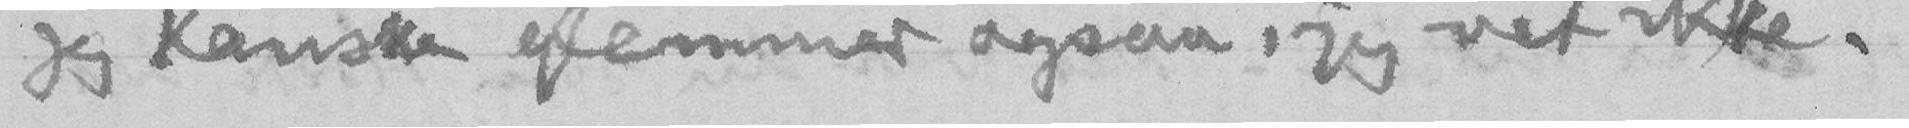jeg kanske glemmer ogsaa, jeg vet ikke.
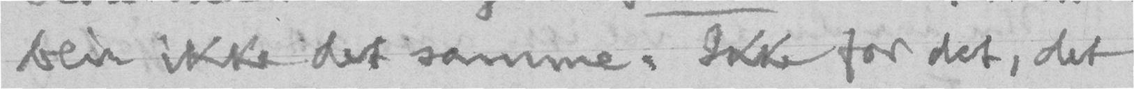blir ikke det samme. Ikke for det, det
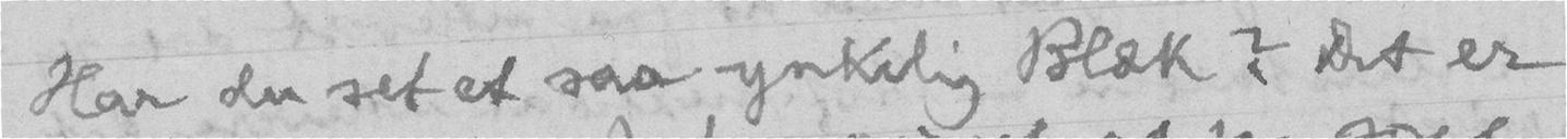Har du set et saa ynkelig Blæk? Det er
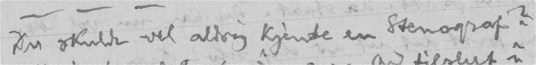Du skulde vel aldrig kjende en Stenograf?
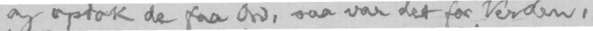og optok de faa Ord, saa var det for Verden,
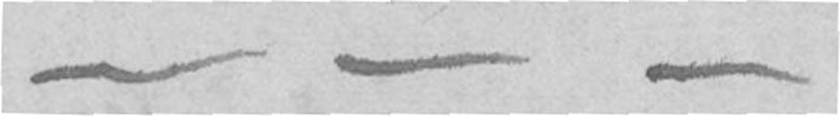- - -
